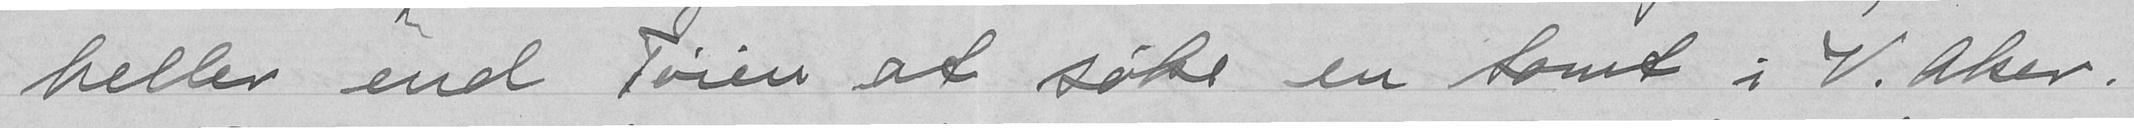heller end Tøien at søke en tomt i V. Aker.
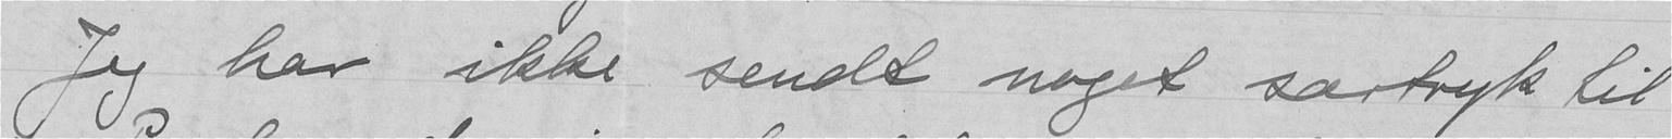Jeg har ikke sende noget særtryk til
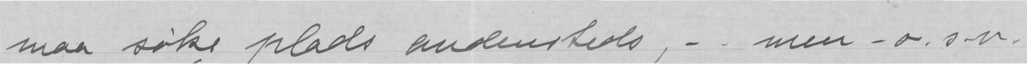maa søke plads andensteds, - men -o. sv.
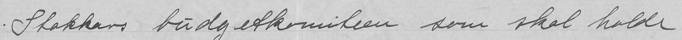Stokkars budgetkomiteen som skal holde
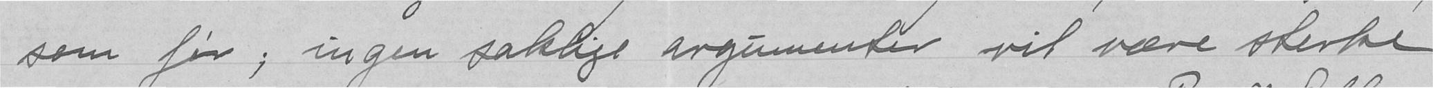som før; ingen saklige argumenter vil være sterke
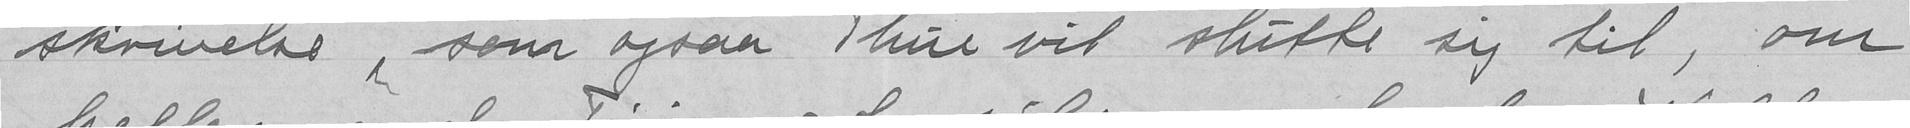skrivelse som ogsaa Thue vil slutte sig til, om
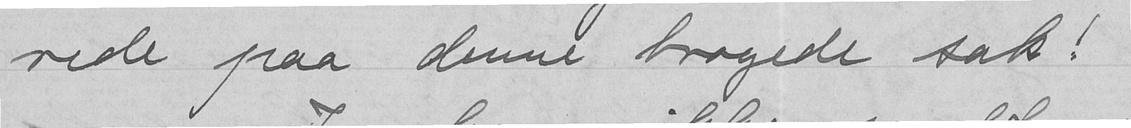rede paa denne brogede sak!
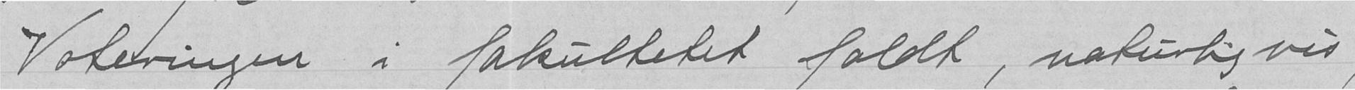Voteringen i fakultetet faldt, naturligvis
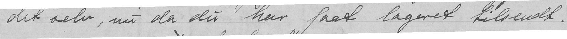det selv, nu da du har faat lageret tilsendt.
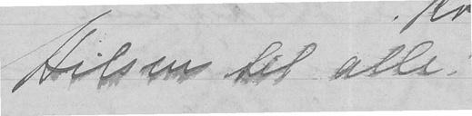Hilsen til alle:
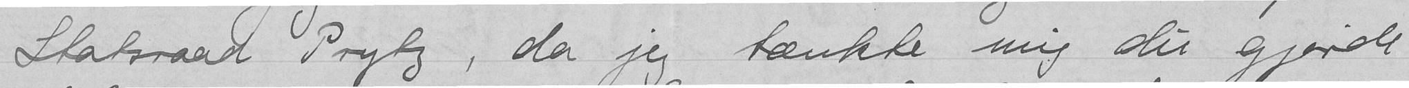Statsraad Prytz, da jeg tænkte mig du gjorde
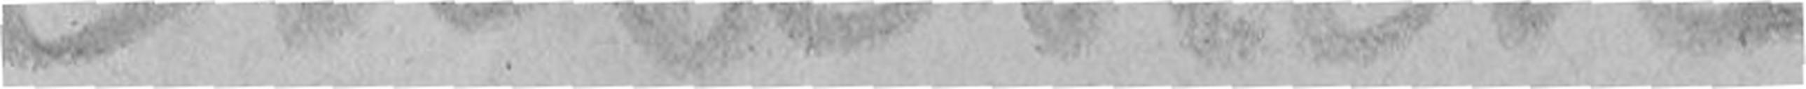E Munch
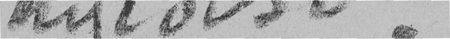hyldest!
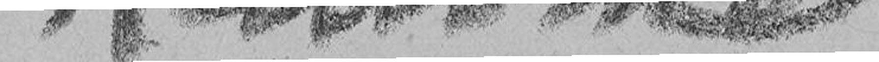Ramme
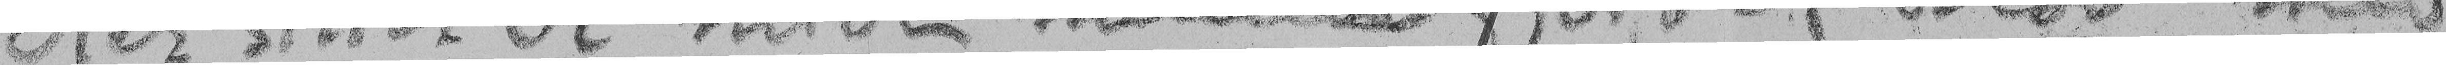Her sitter vi midt mellem fjord og skov med
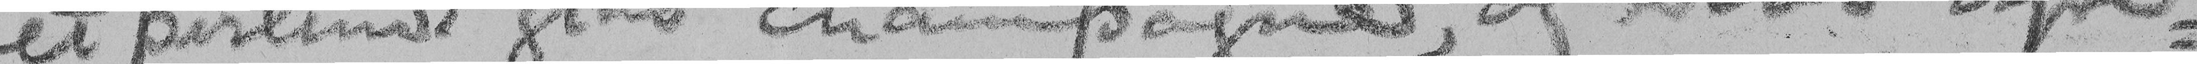et perlende glas champagne, og Deres dyre-
